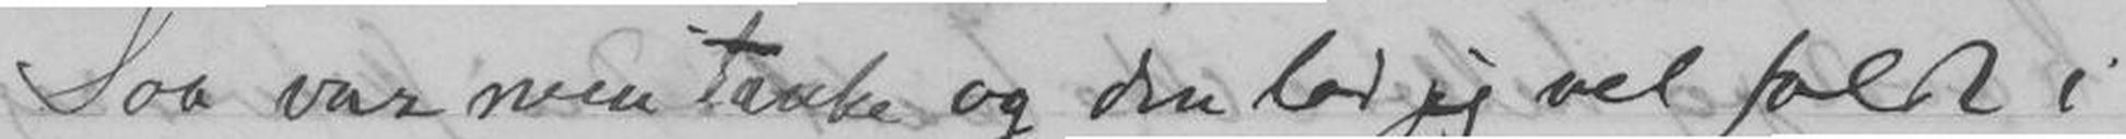Saa var min tanke og den lod jeg vel falde i
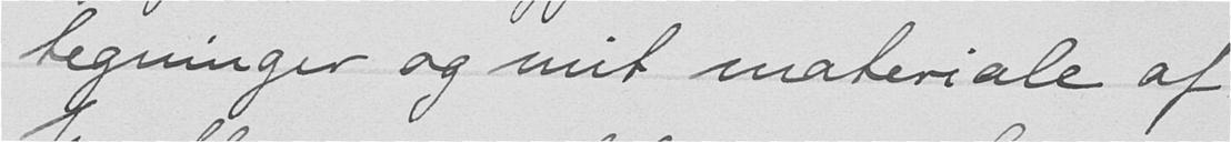tegninger og mit materiale af
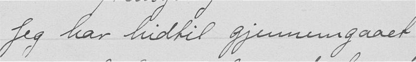Jeg har hidtil gjennemgaaet
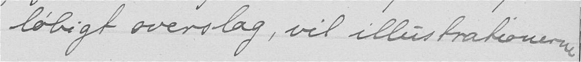løbigt overslag, vil illustrationerne
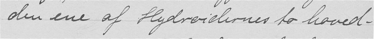den ene af Hydroidernes to hoved-
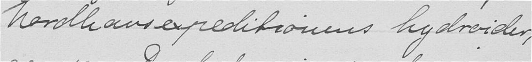Nordhavsexpeditionens hydroider,
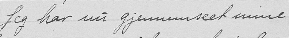Jeg har nu gjennemseet mine
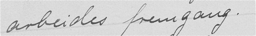arbeides fremgang.
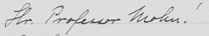Hr. Professor Mohn!
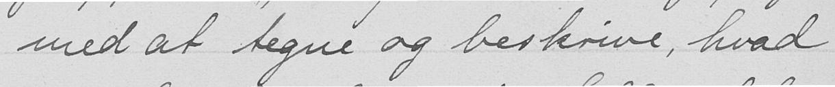med at tegne og beskrive, hvad
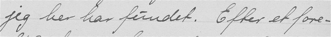jeg her har fundet. Efter et fore-
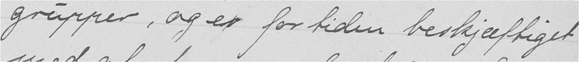grupper, og er for tiden beskjæftiget
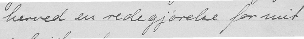herved en redegjørelse for mit
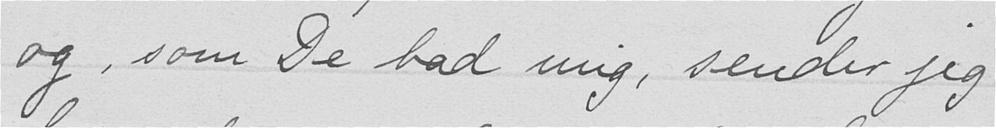og, som De bad mig, sender jeg
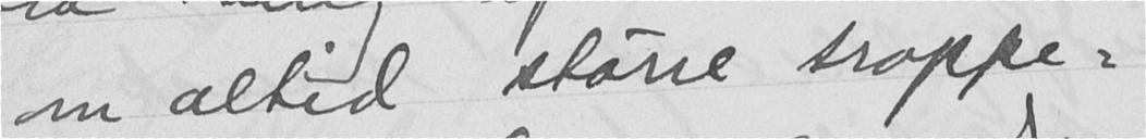om altid større troppe-
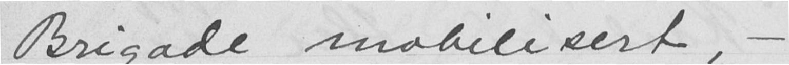Brigade mobilisert, -
